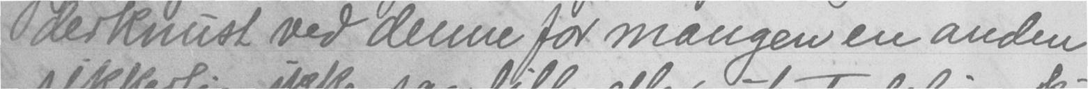derknust ved denne for mangen en anden
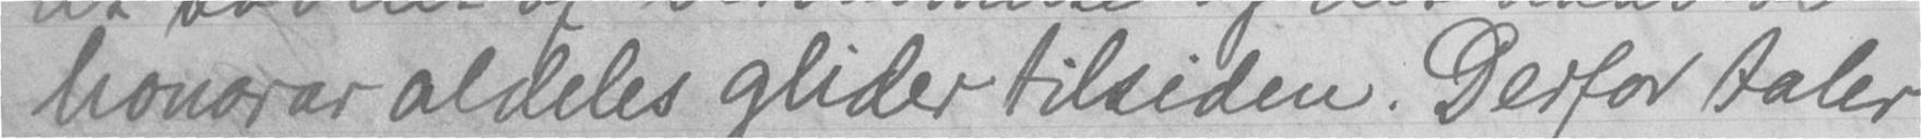honorar aldeles glider tilsiden. Derfor taler
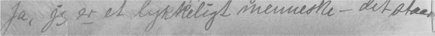Ja, jeg er et lykkeligt menneske - det staar
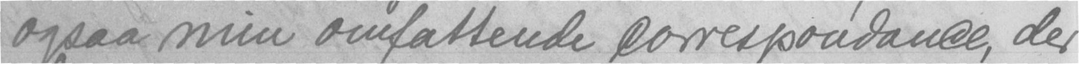ogsaa min omfattende correspondance, der
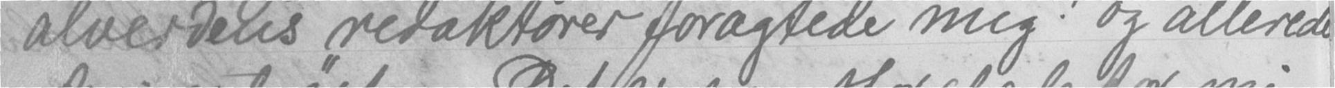alverdens redaktører foragtede mig! og allerede
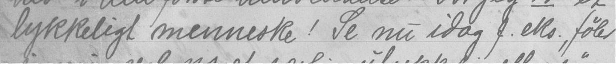lykkeligt menneske! Se nu idag f. eks., føler
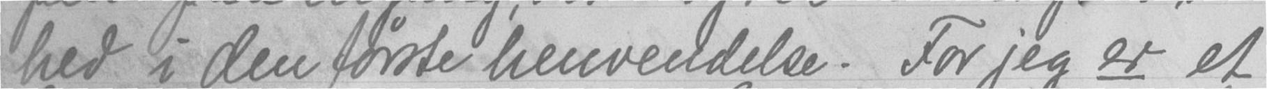hed i den første henvendelse. For jeg er et
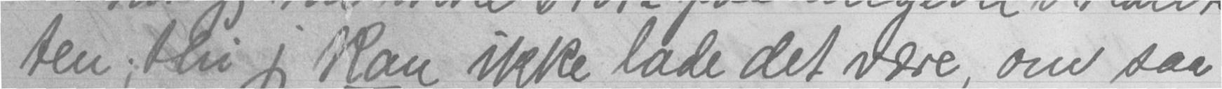ten; thi jeg kan ikke lade det være, om saa
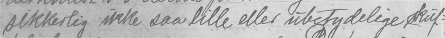sikkerlig ikke saa lille eller ubetydelige skuf-
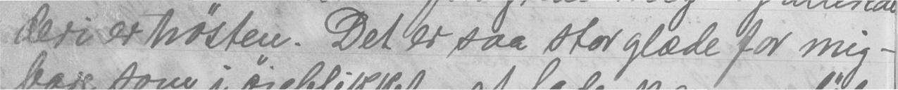deri er trøsten. Det er saa stor glæde for mig -
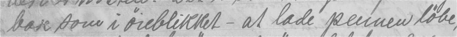bare som i øieblikket - at lade pennen løbe,
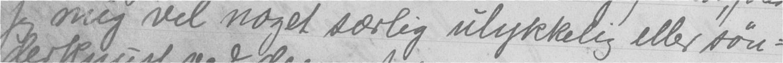jeg mig vel noget særlig ulykkelig eller søn-
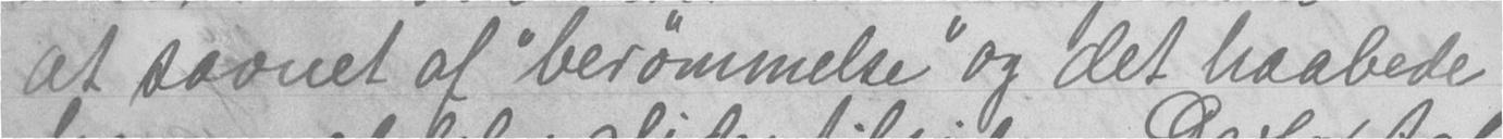at savnet af "berømmelse" og det haabede
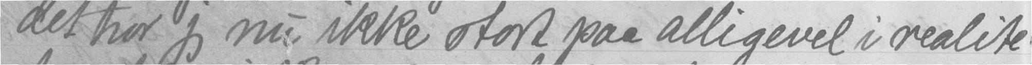det tror jeg nu ikke stort paa alligevel i realite-
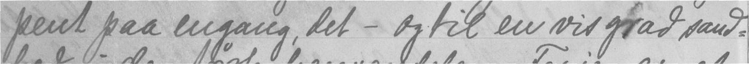pent paa engang, det - og til en vis grad sand-
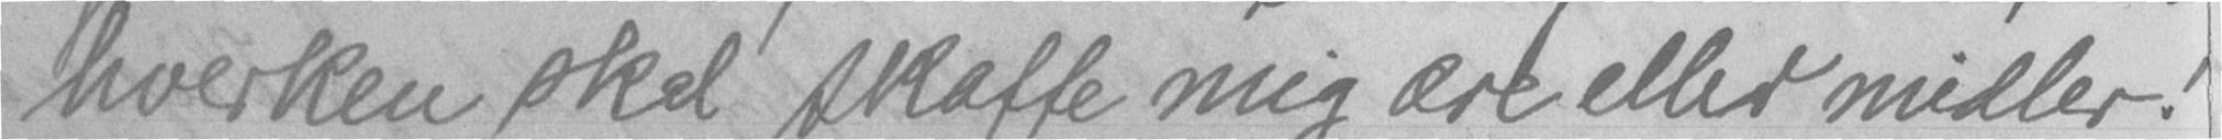hverken skal skaffe mig ære eller midler!
